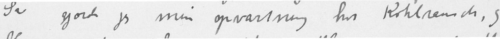Saa gjorde jeg min opvartning hos Kohlrausch, og
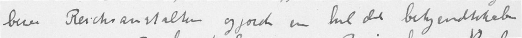beser Reichsanstalten gjorde en hel del bekjendtskaber
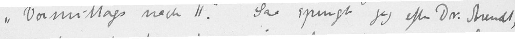"Vormittags nach 11." Saa spurgte jeg efter Dr. Arendt,
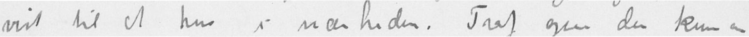vist til et hus i nærheden. Traf ogsaa der kun en
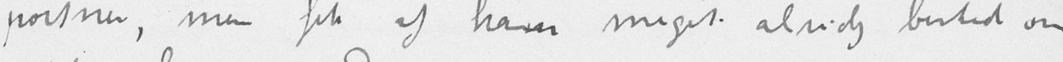portner, men fik af ham meget alsidig besked om
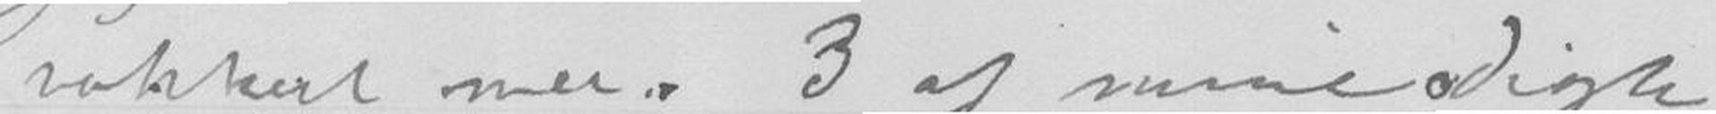vakkert mer. 3 af mine Digte
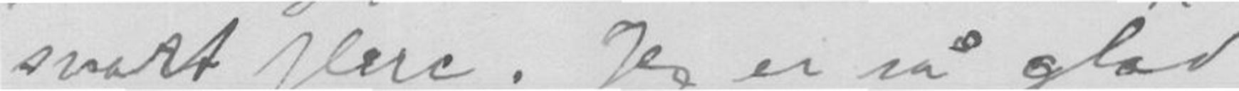snart flere. Jeg er så glad
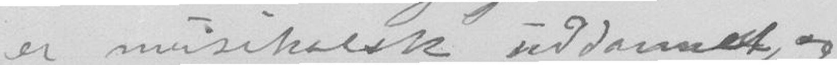er musikalsk uddannet, og
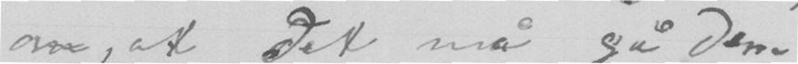om, at Det må gå Dem
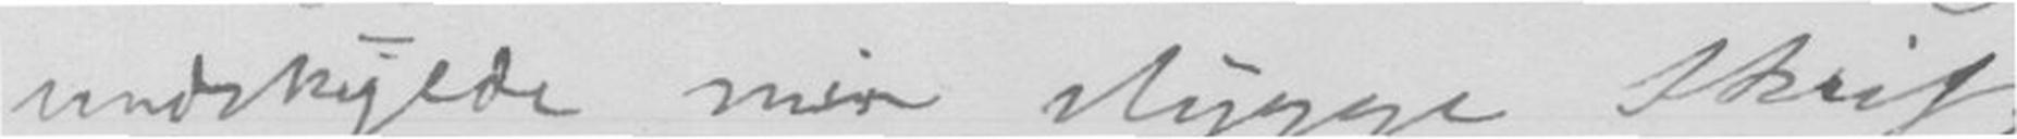undskylde min stygge skrift
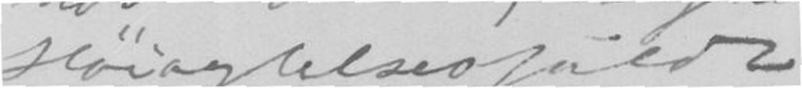Høiagtelsesfuldt
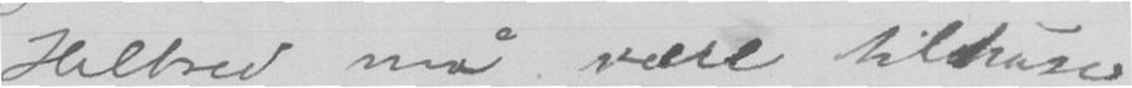Helbred må være tilhuse
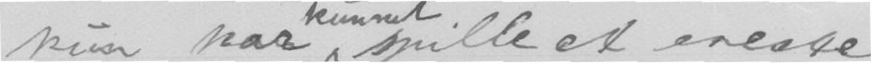kun har spille et eneste
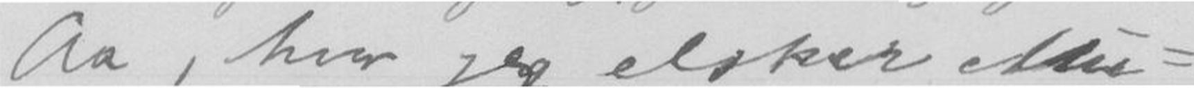Aa, hvor jeg elsker Mu-
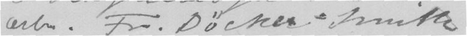Ærb. Fr Döcker=Smith
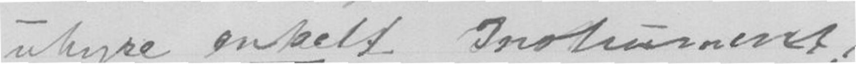uhyre enkelt Instrument!, -
